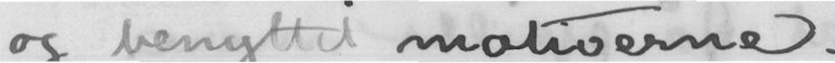og benyttet motiverne.
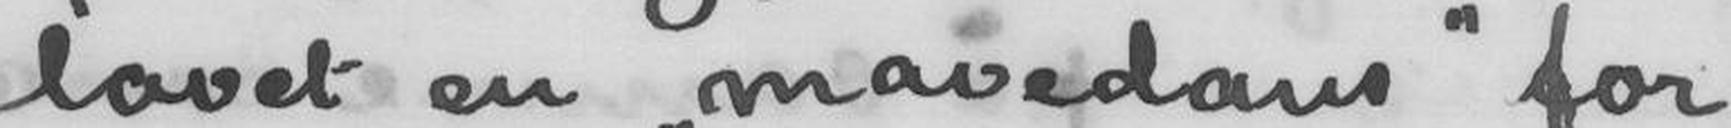lavet en mavedans for
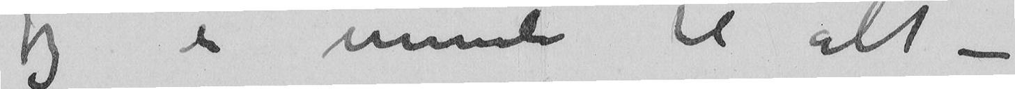jeg er umuli til alt –
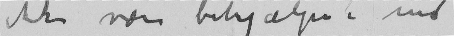ikke være behjælpeli med
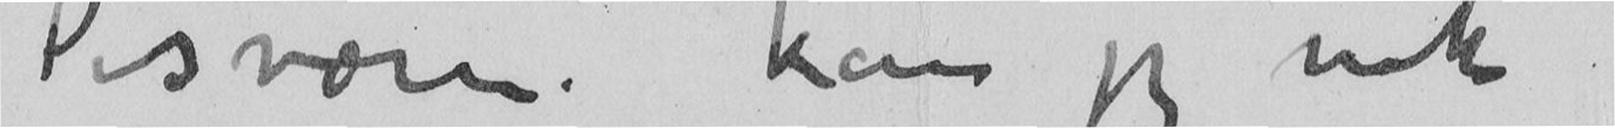Desværre kan jeg nok
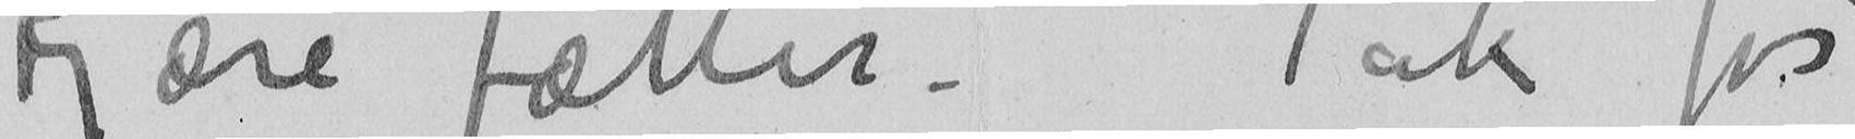Kjære fætter! Tak for
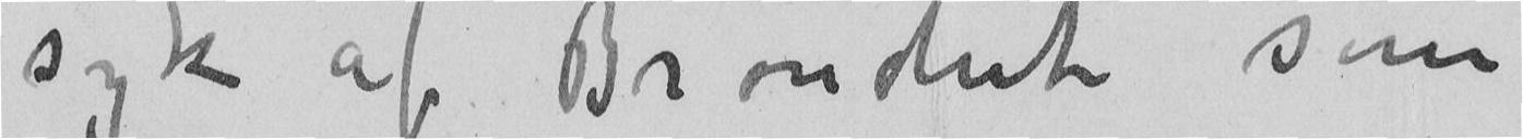syk af Bronchit som
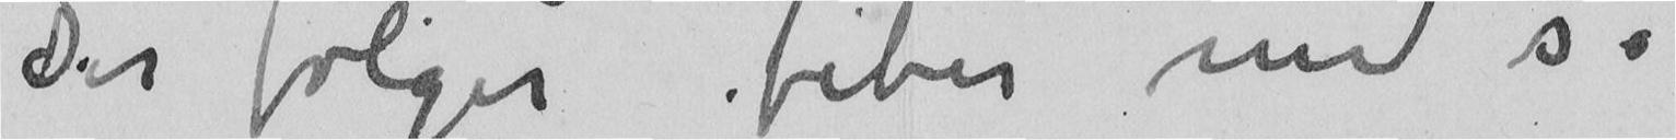Der følger feber med så
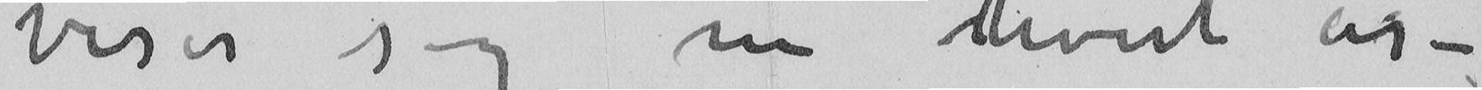viser sig nu hvert år –
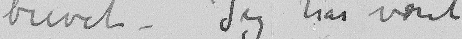brevet – Jeg har været
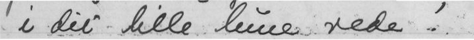i dit lille lune rede!
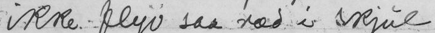ikke flyv saa ræd i skjul,
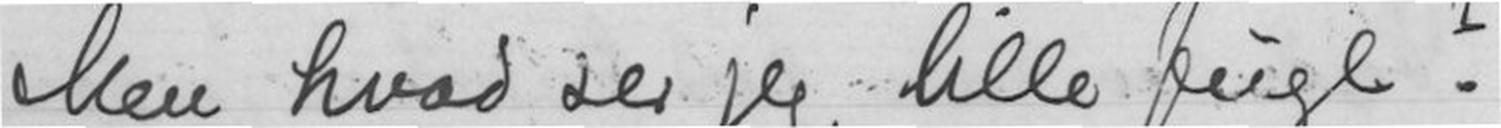Men hvad ser jeg lille fugl?
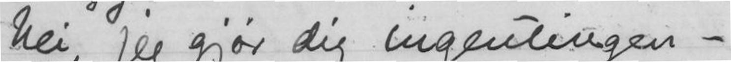Nei, jeg gjør dig ingentingen -
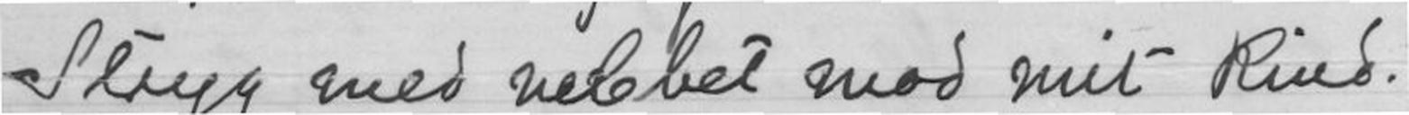Stryg med nebbet mod mit kind!
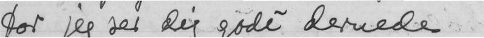for jeg ser Dig godt dernede
